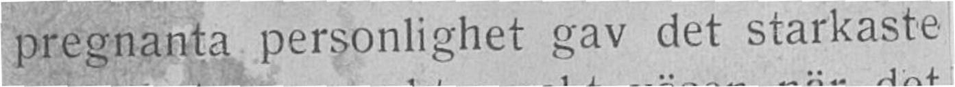pregnant personlighet gav det starkaste
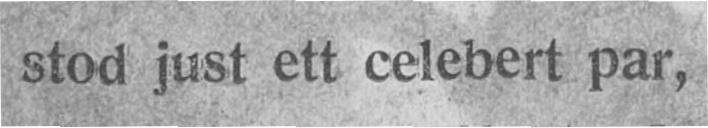stod just ett celebert par,
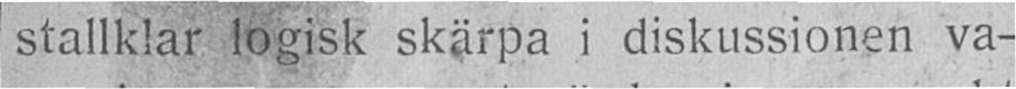stallklar logisk skärpa i diskussionen va-
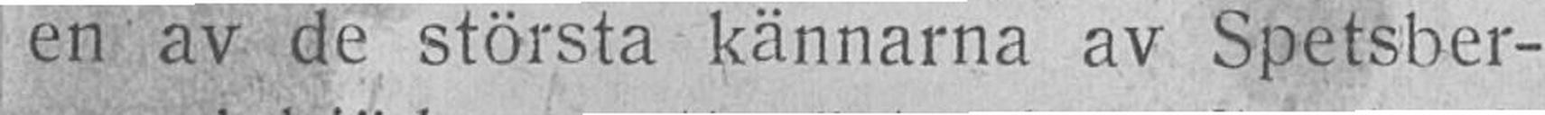en av de största kännarna av Spetsber-
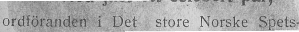ordföranden i Det store Norske Spets-
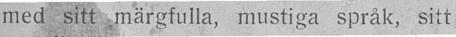med sitt märgfulla, mustiga spräk, sitt
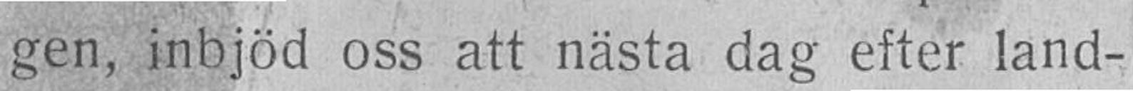gen, inbjöd oss att nästa dag efter land-
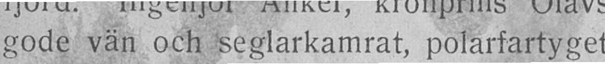gode vän och seglarkamrat, polarfartyget
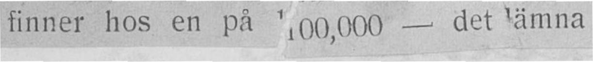finner hos en på 100,000 - det lämna
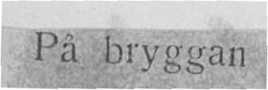På bryggan
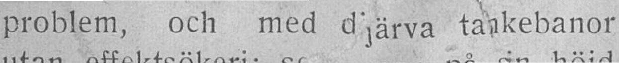problem, och med djärva tankebanor
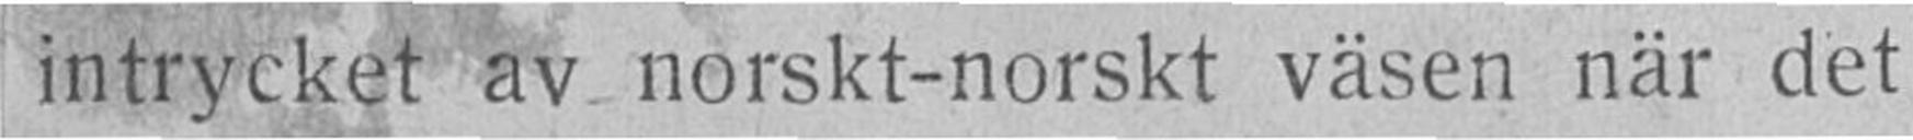intrycket av norskt-norskt väsen när det
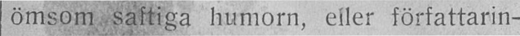ömsom saftiga humorn, eller författarin-
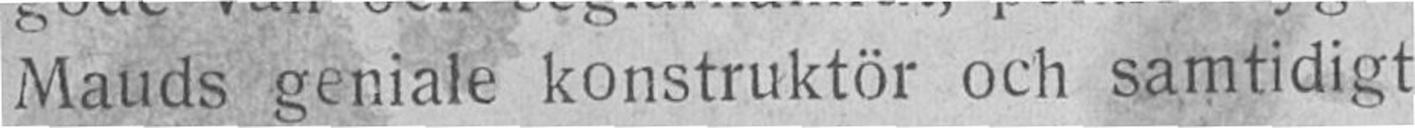Mauds geniale konstruktör och samtidigt
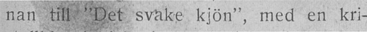nan till Det svake kjön", med en kri-
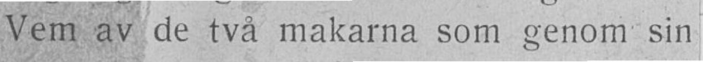Vem av de två makarna som genom sin
